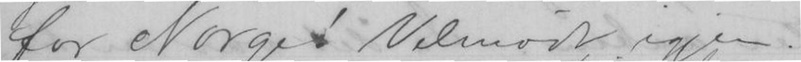for Norge! Velmødt igjen!
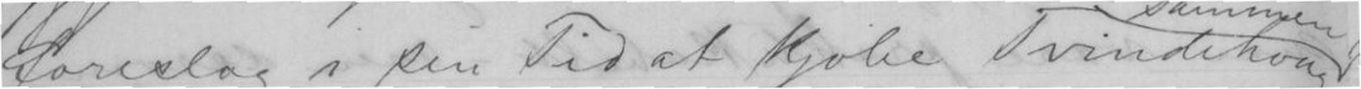foreslog i sin Tid at Kjøbe Tvindehoug
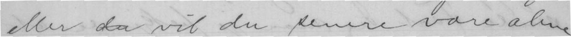eller du vil du senere være alene
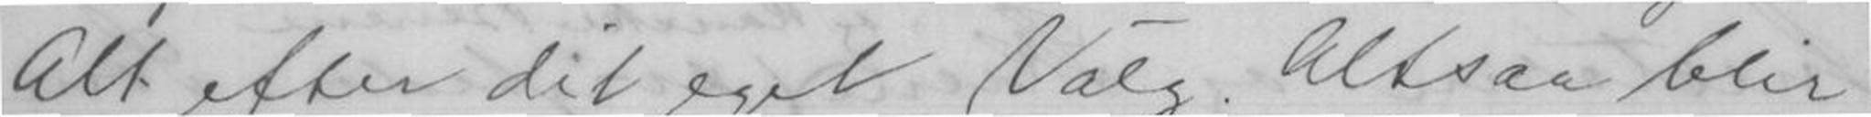Alt efter dit eget Valg. Altsaa blir
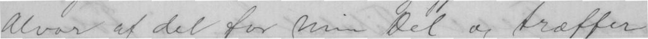Alvor af det for min Del og træffer
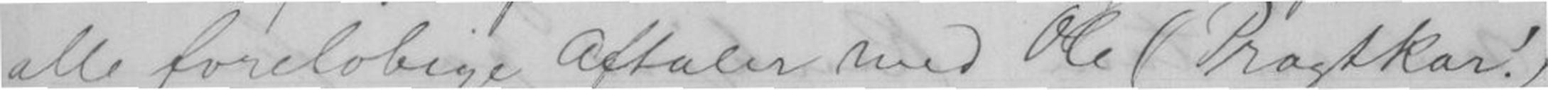alle foreløbige Aftaler med Ole (Pragtkar!)
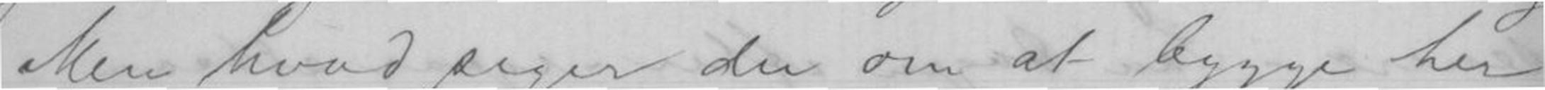Men hvad siger du om at bygge her
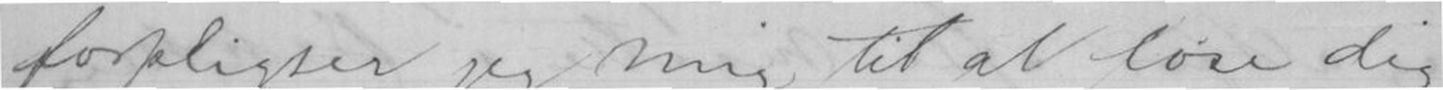forpligter jeg mig til at løse dig
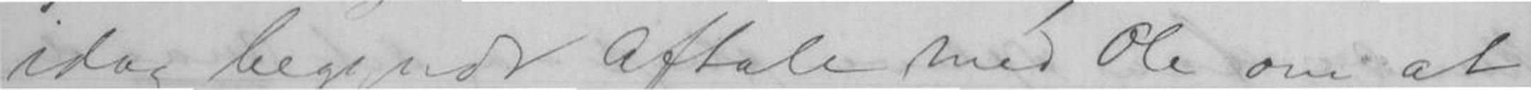idag begyndt Aftale med Ole om at
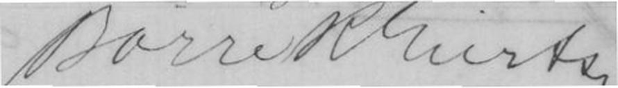Børre R Giertsen
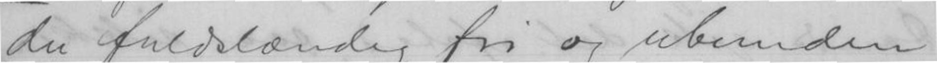du fuldstændig fri og ubunden
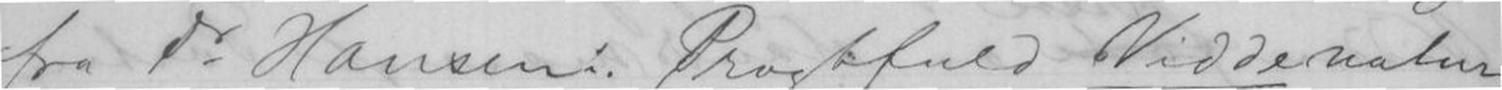fra dr Hansens Pragtfuld Viddenatur
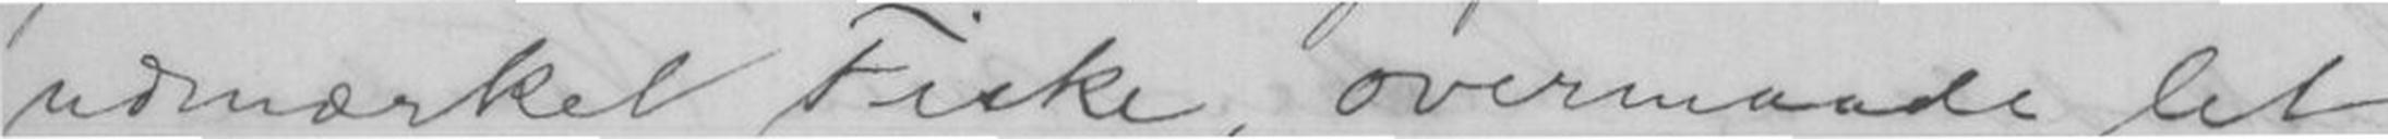ufmærket Fiske, overmaade let
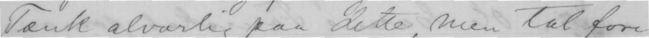Tænk alvorlig paa dette, men tal fore
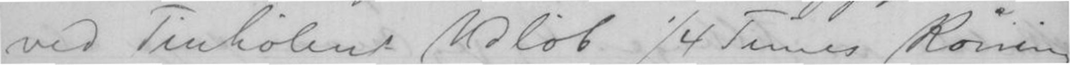ved Tinhølens Udløb ¼ Times Køring
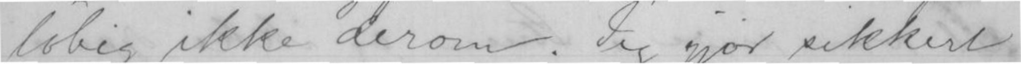løbig ikke derom. Jeg gjør sikkert
